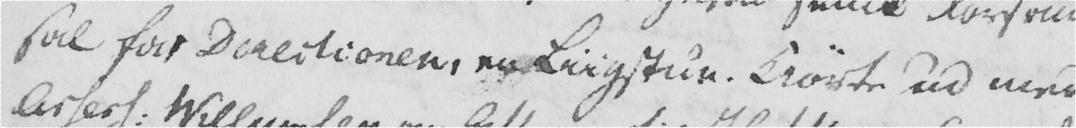sal for Directionen, en Liigstue. Kiørte ud med
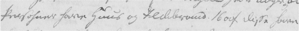Personer have Huus og Ildebrand. 16 af disse have
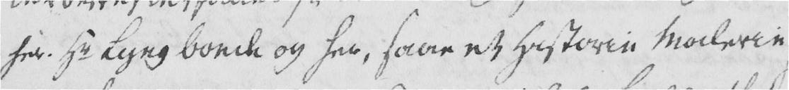her. Hr Lyng boede og her, saae et Historie Malerie
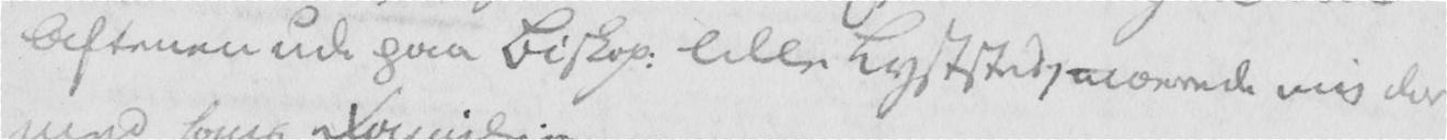Aftenen ude paa Biskop. lille Lyststed, morede mig der
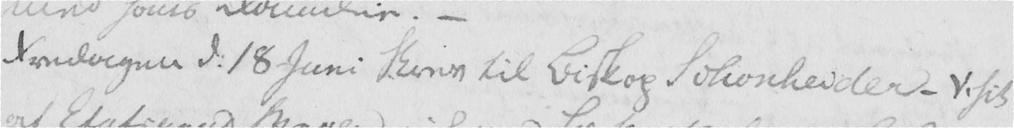Fredagen d. 18 Juni Skriv til Biskop Schønheider - Visit
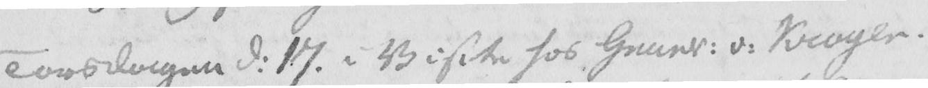Torsdagen d. 17. i Visite hos Gener. v. Krogen.
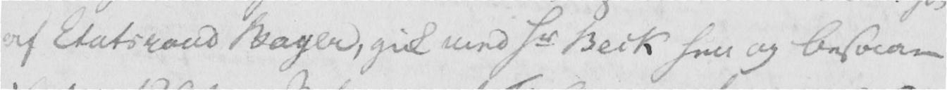af Etatsraad Bayer, gik med Hr Beck hen og besaae
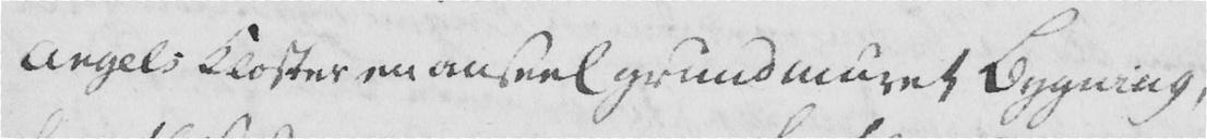Angels Kloster en anseel grundmuret Bygning,
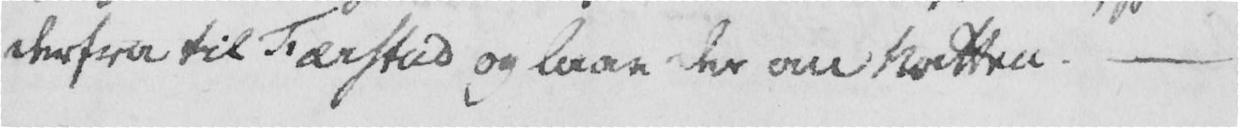derfra til Færstad og laae der om Natten. -
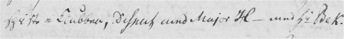spiste i Klubben, Disput med Major H - med Hr Beck.
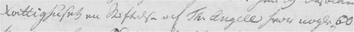Fattighuset en Stiftelse af Th. Angell hvor nogle 60
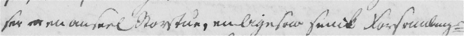ser er en anseel Storstue, en ligesaa smuck Forsamlings-
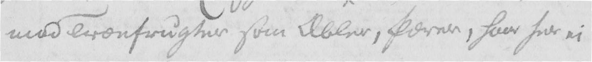mod Træefrugter som Æbler, Pærer, har her ei
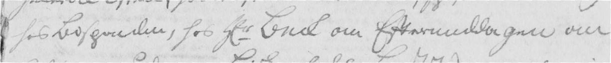hos Bispinden, hos Hr Beck om Eftermiddagen om
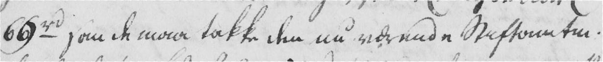69 rd som de maae takke den nu værende Stiftamtm.
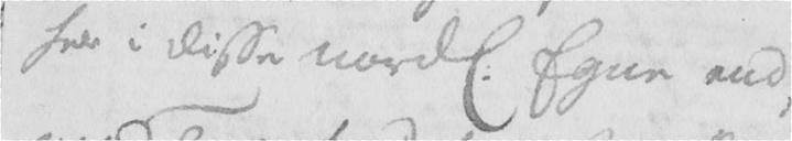her i disse nordl. Egne end
